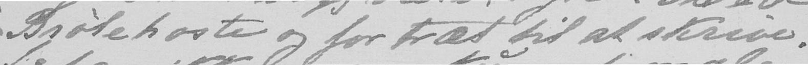Brølehoste og for træt til at skrive.
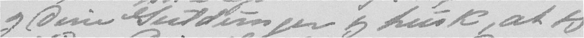og Dine Guldunger og husk, at jeg
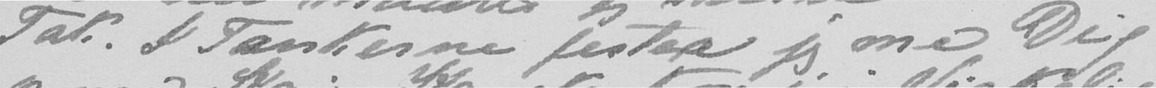Tak. I Tankerne fester jeg med Dig
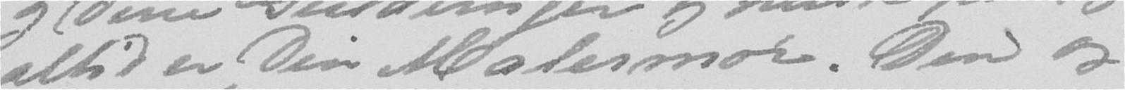altid er Din Malermøi. Din og
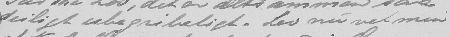deiligt ubegribeligt. Lev nu vel min
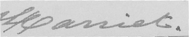Harriet.
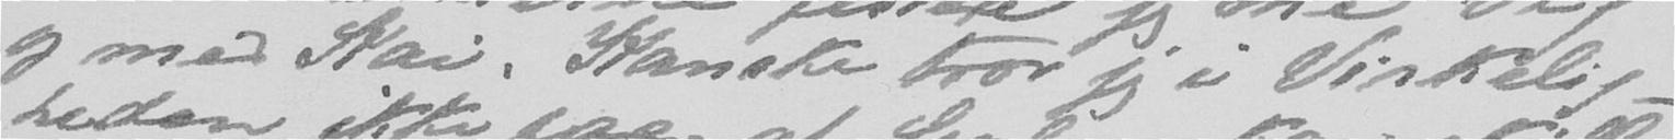og med Kai. Kanske tror jeg i Virkelig-
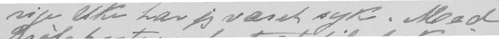rige Uke har jeg været syk. Med
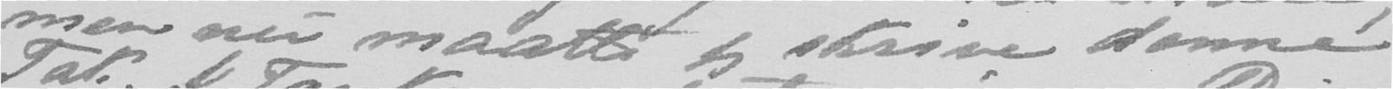men nu maatte jeg skrive denne
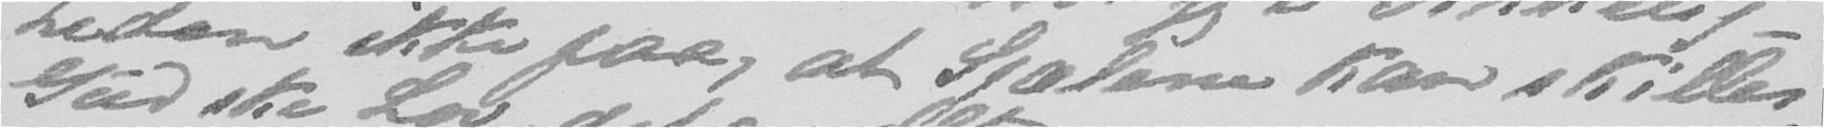heden ikke paa, at Sjælene kan skilles.
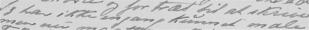Jeg har ikke engang kunnet male;
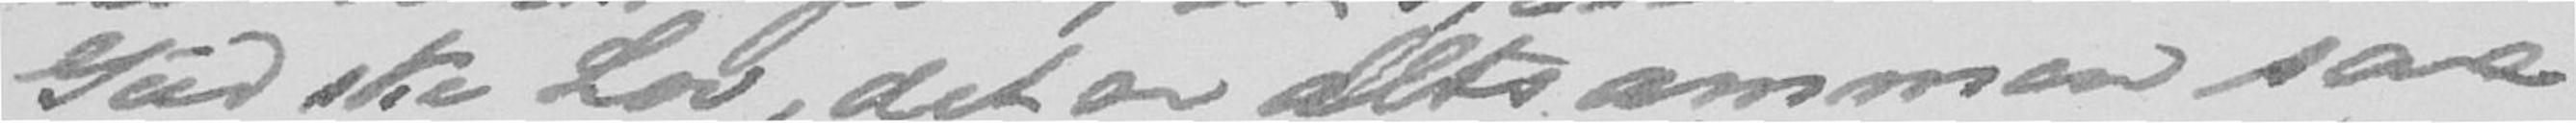Gud ske Lov, det er altsammen saa
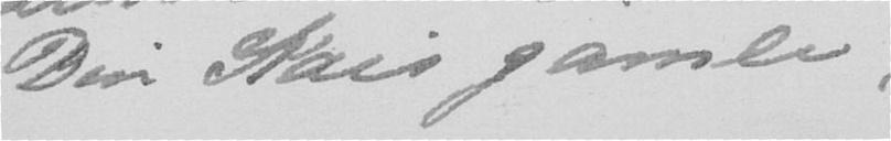Din Kais gamle
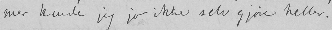mer kunde jeg jo ikke selv gjøre heller.
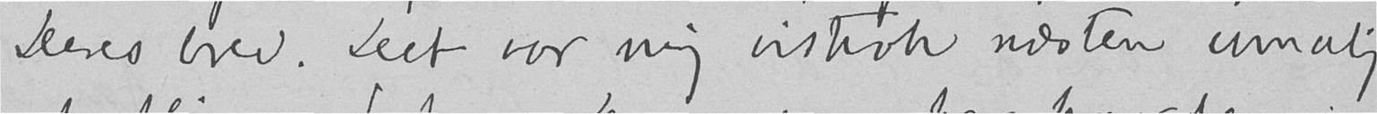Deres brev. Det var mig vistnok næsten umulig
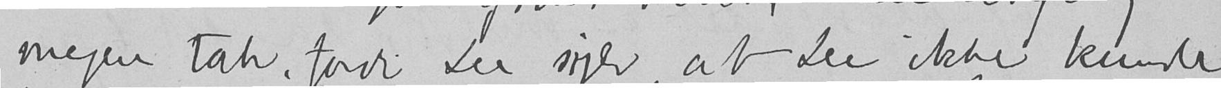megen tak, fordi De siger, at De ikke kunde
